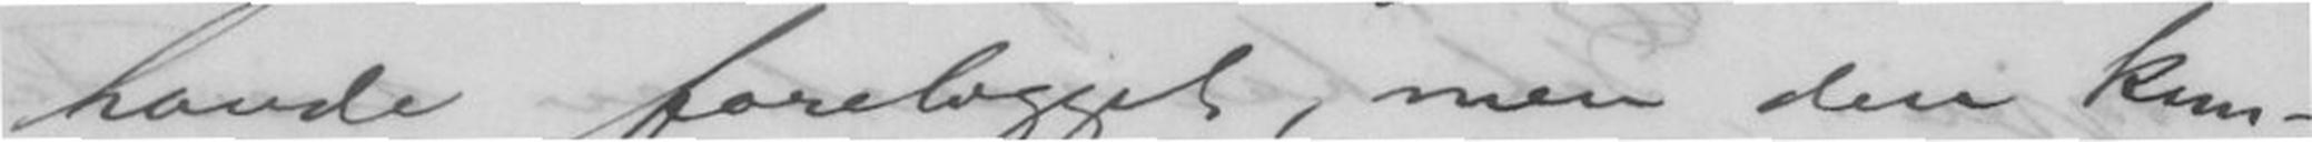havde foreligget, men den kun-
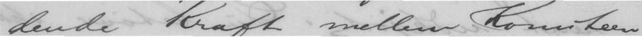dende Kraft mellem Komiteen
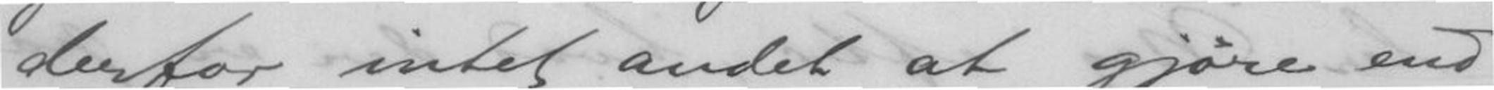derfor intet andet at gjøre end
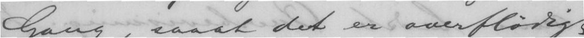Gang, saaat det er overflødigt
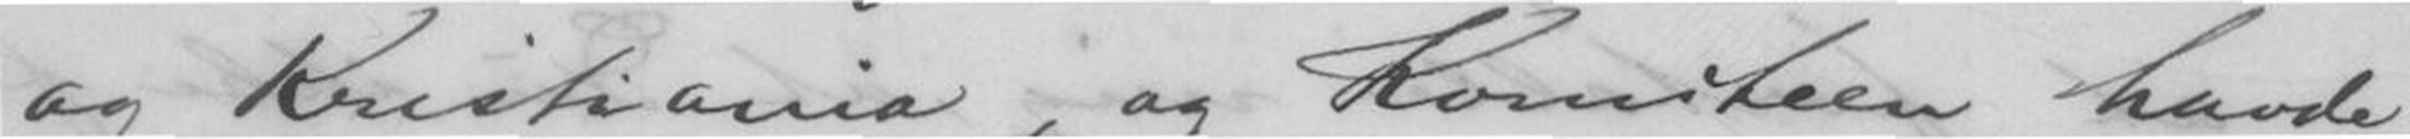og Kristiania, og Komiteen havde
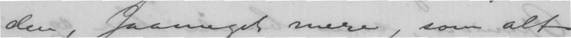den, saameget mere, som alt
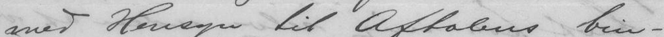med Hensyn til Aftalens bin-
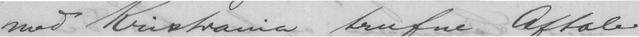med Kristiania trufne Aftale
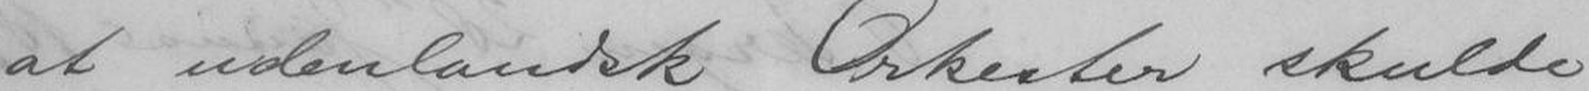at udenlandsk Orkester skulde
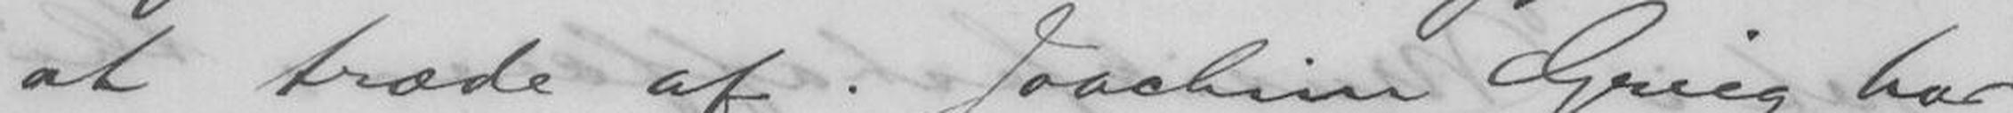at træde af. Joachim Grieg har
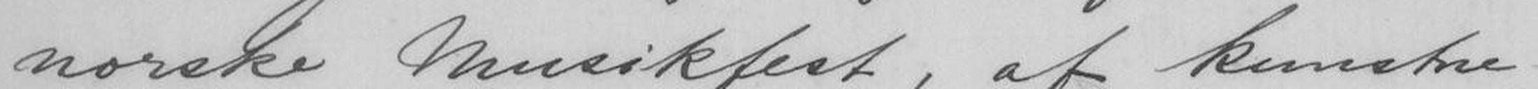norske Musikfest, af kunstne-
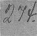274.
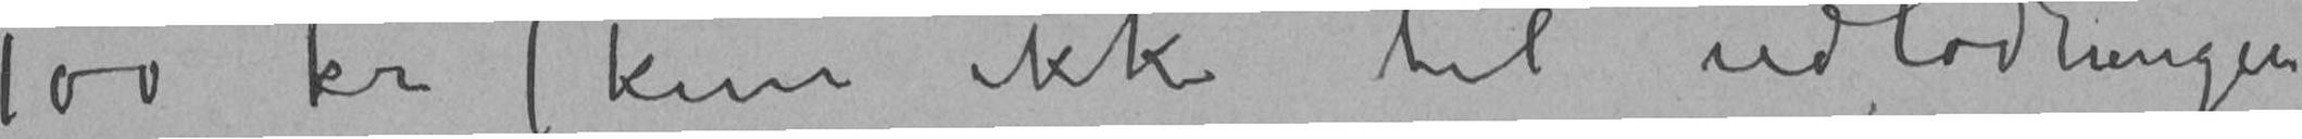100 kr (kun ikke til udlodningen
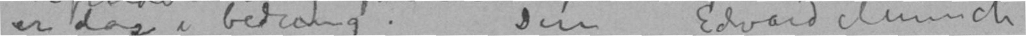er dog i bedring. Din Edvard Munch
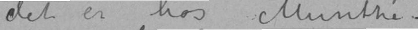det er hos Munthe –
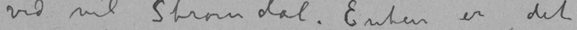ved vel Strømdal. Enten er det
